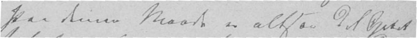Paa denne Maade er altsaa det Gebet
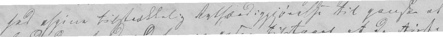hed afgive tilstrækkelig Retfærdiggjørelse til ganske at
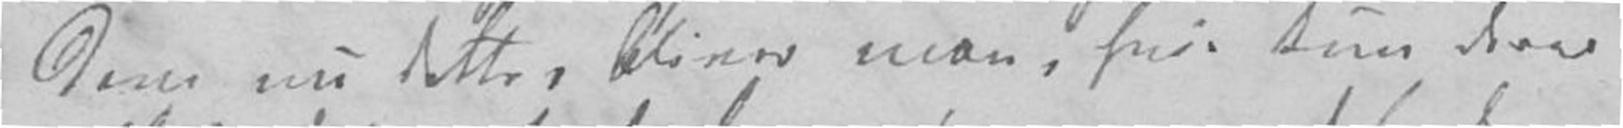dem nu dette, bliver man, hvis kun deres
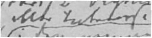eller Interesse
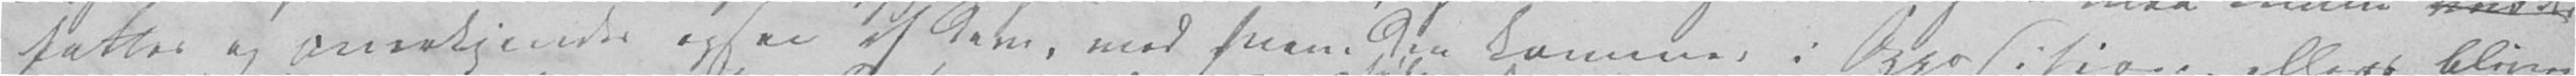fattes og anerkjendes ogsaa af dem, med hvem den kommer i Opposition, ellers bliver
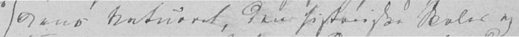dens Naturret, den historiske Skoles og
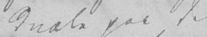dvæle paa de
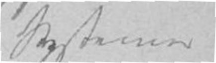Systemer
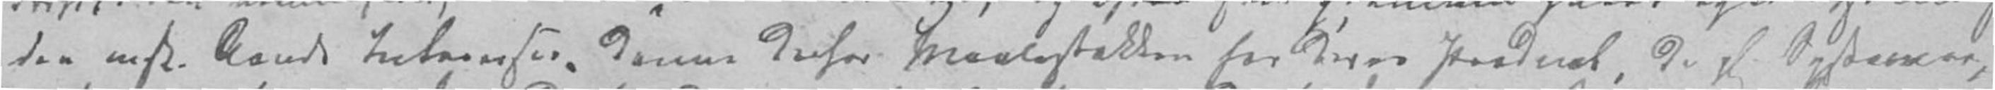den msk. Aands Interesser danner derfor Maalestokken for deres Product, de fl. Systemer,
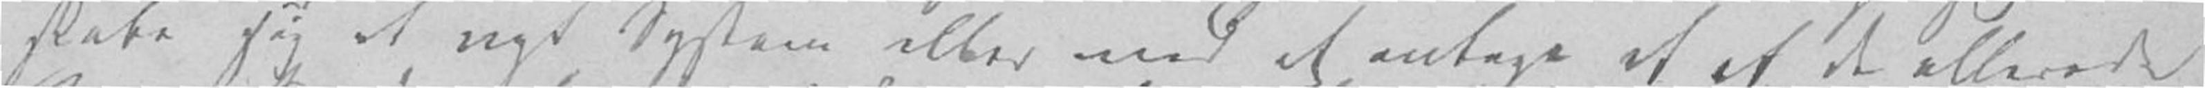skabe sig et nyt System eller med at antage et af de allerede
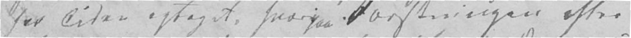for Tiden optaget, hvorpaa Forskningen efter
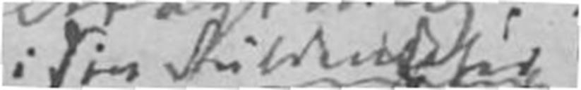i sin Fuldendthed
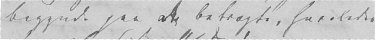begynde paa at betragte, hvorledes
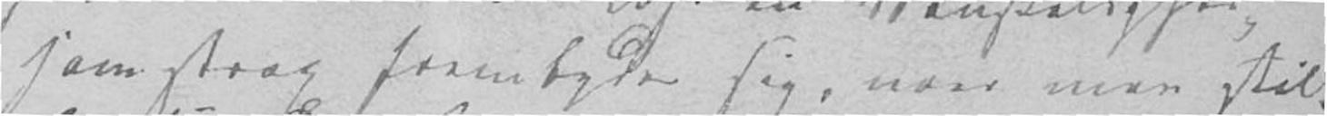som strax frembyder sig, naar man stil-
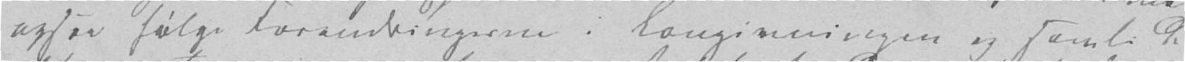ogsaa følge Forandringerne i Lovgivningen og samle de
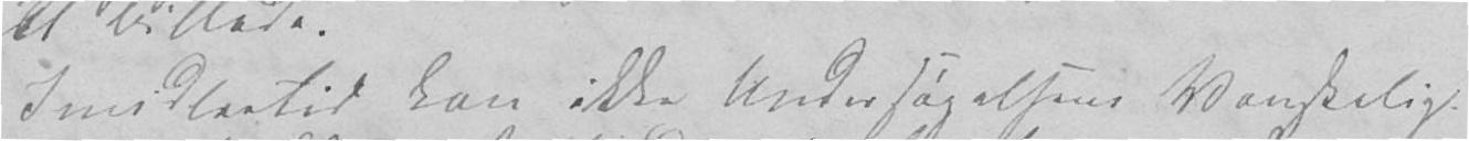Imidlertid kan ikke Undersøgelsens Vanskelig-
